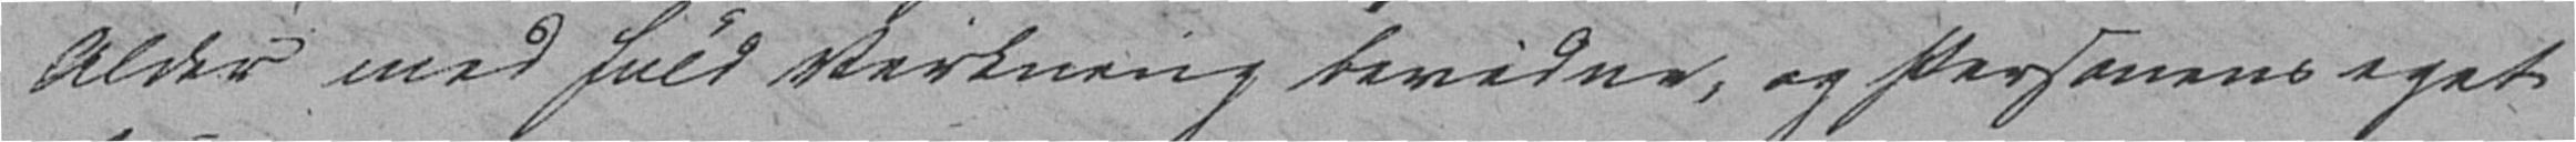Alder med fuld Virkning bevidne, og Personens eget
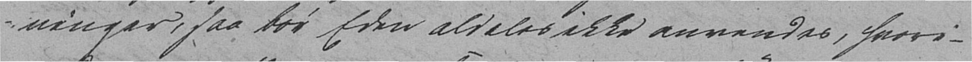ninger, saa bør Eden aldeles ikke anvendes, hvori-
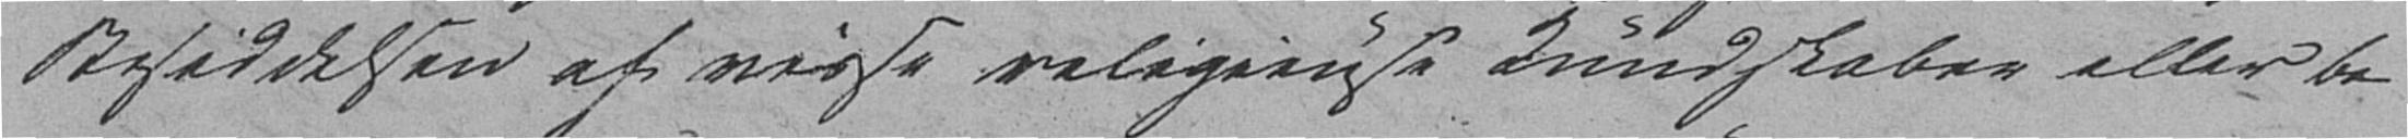Besiddelsen af visse religieuse Kundskaber eller be-
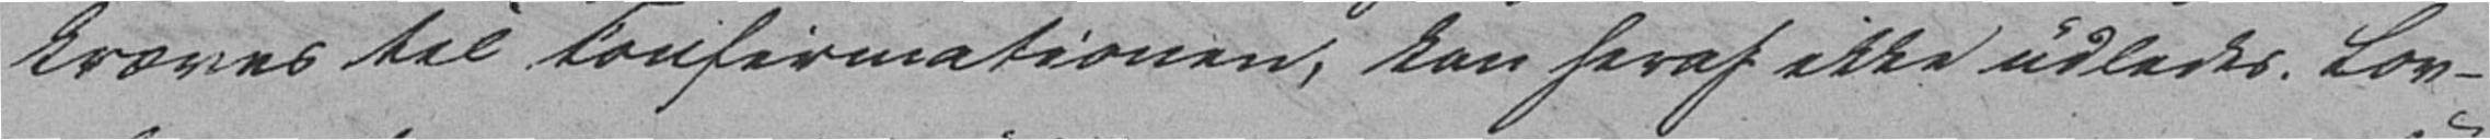kræves til Konfirmationen, kan heraf ikke udledes. Lov-
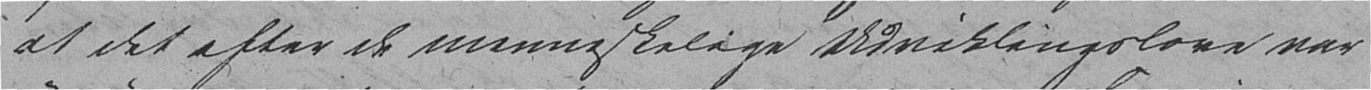at det efter de menneskelige Udviklingslove var
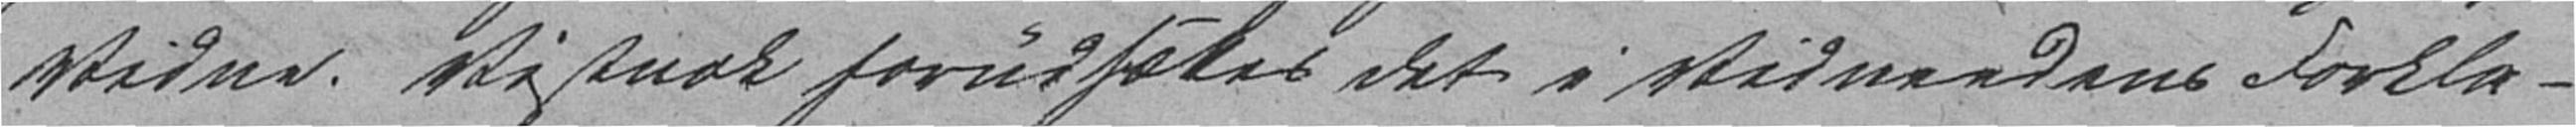Vidne. Vistnok forudsættes det i Vidneedens Forkla-
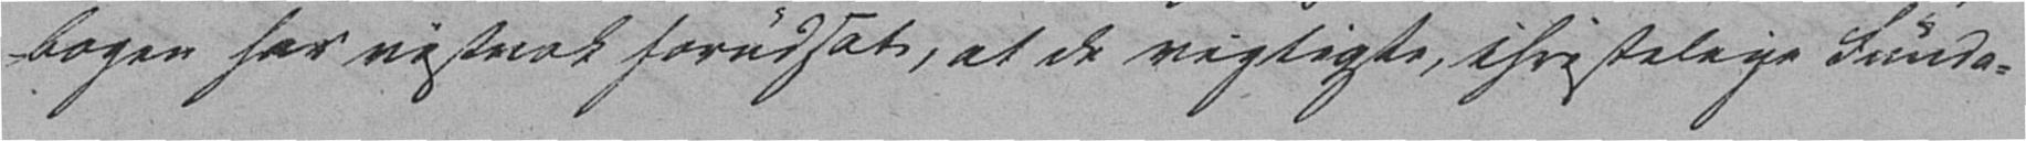bogen har vistnok forudsat, at de vigtigste, christelige Funda-
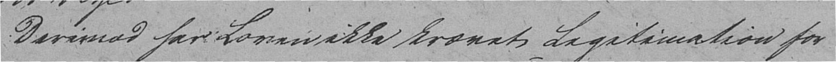Derimod har Loven ikke krævet Legitimation for
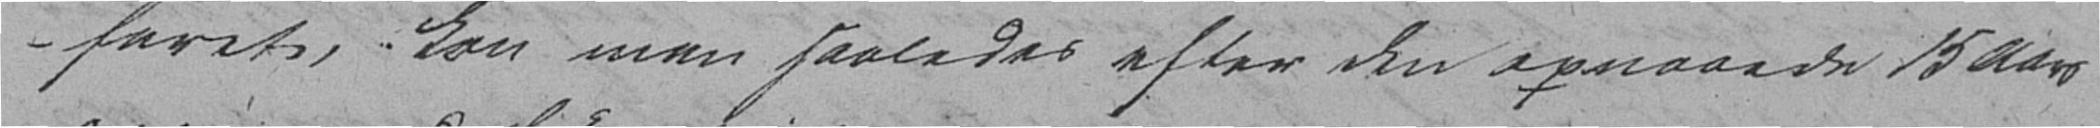faret, kan man saaledes efter den opnaaede 15 Aars
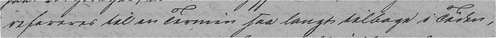refereres til en Termin saa langt tilbage i Tiden,
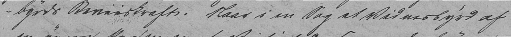byrds Beviiskraft. Naar i en Sag et Vidnesbyrd af
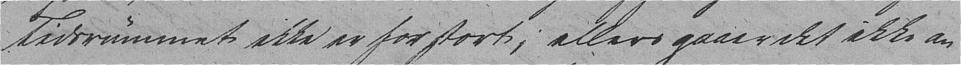Tidsrummet ikke er for stort, ellers gaaer det ikke an
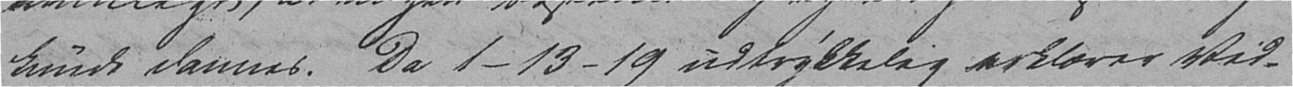kunde dannes. Da 1-13-19 udtrykkelig erklærer Vid-
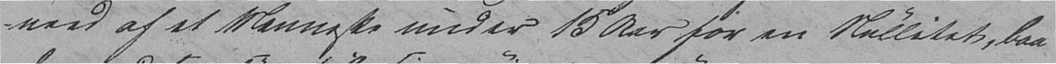need af et Menneske under 15 Aar for en Nullitet, baa-
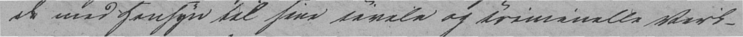de med Hensyn til sine civile og criminelle Virk-
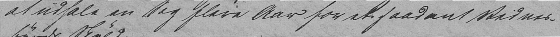at udhale en Sag flere Aar for et saadant Vidnes-
